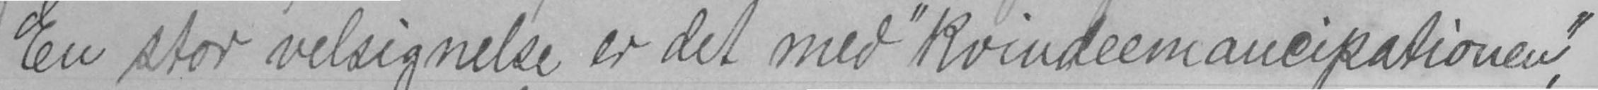En stor velsignelse er det med "kvindeemancipationen",
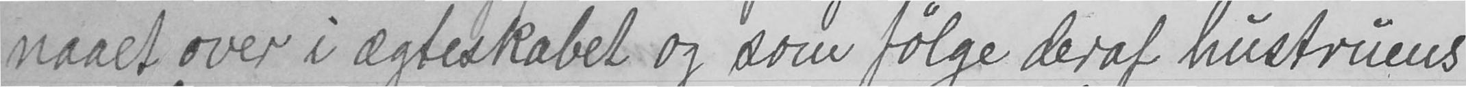naaet over i ægteskabet og som følge deraf hustruens
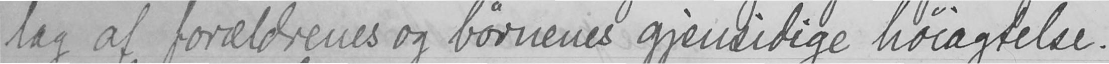lag af forældrenes og børnenes gjensidige høiagtelse.
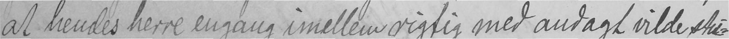at hendes herre engang imellem rigtig med andagt vilde stu-
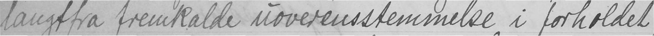langtfra fremkalde uoverensstemmelse i forholdet,
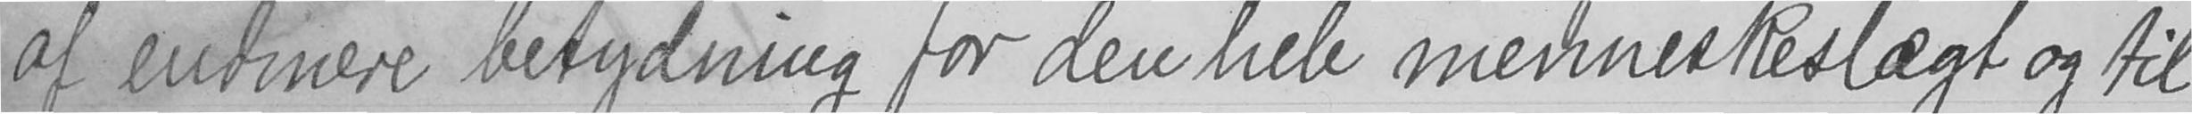af endmere betydning for den hele menneskeslægt og til
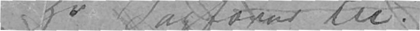Hr Sagfører Lie!
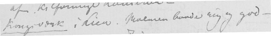Kongsværk i Lier. Malmen baade ny og god -
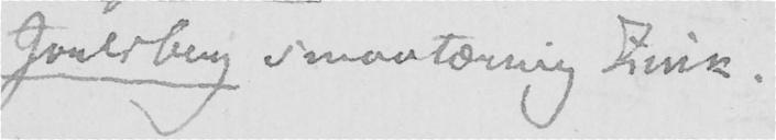Jarlsberg smaatæring Zink.
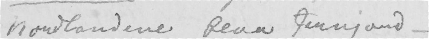Nordlandene Rena Innjord
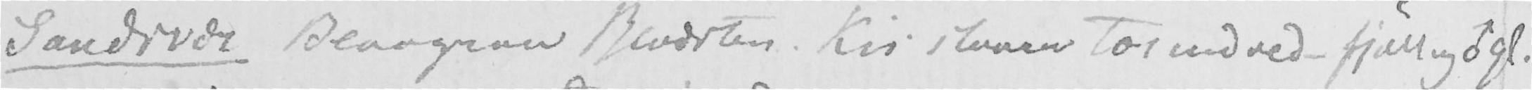Sandsvær Blaagraa Blofsten. Kisstenen tor ned ved - fjall og ♂ gl.
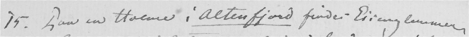15. Paa en Holme i Altenfjord findes Eisenglimmer
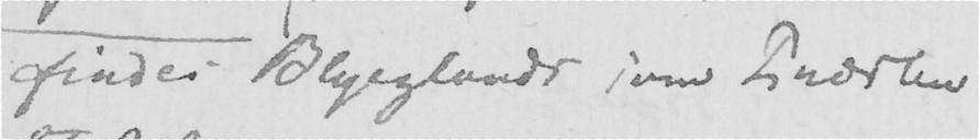findes Blyeglands som Puærlen
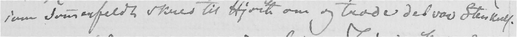som Somerfeldt skues til Hjorth om og trode det var Stenkals.
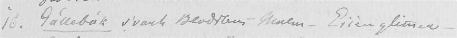16. Gällebäk svart Blodsten-Malm - Eisenglimer -
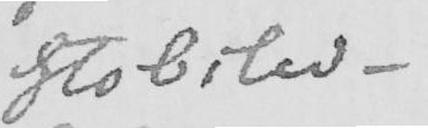Stobslen -
